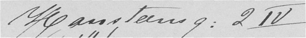Hansteens g. 2 IV
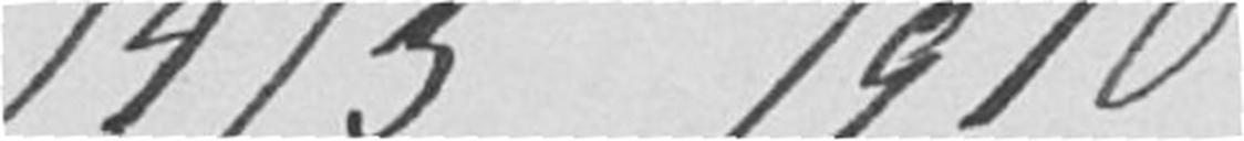14/3 1910
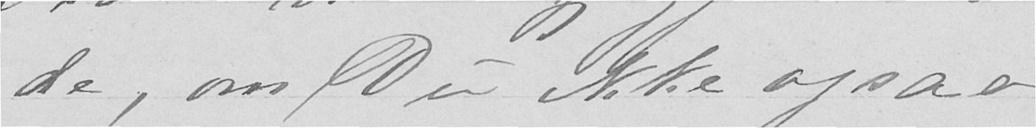de, om Du ikke ogsaa
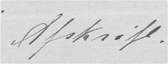Afskrift.
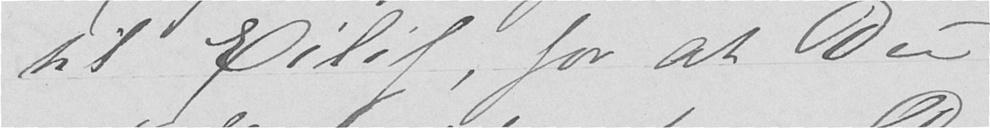til Eilif, for at Du
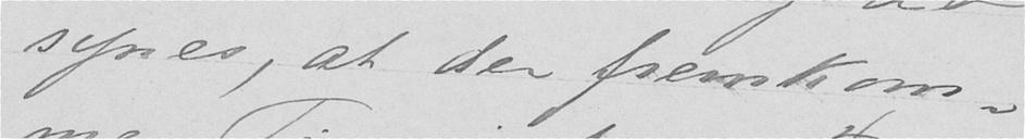synes, at der fremkom-
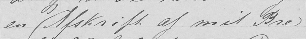en Afskrift af mit Brev
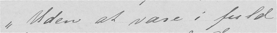"Uden at være i fuld
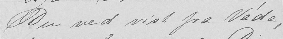Du ved vist fra Véda,
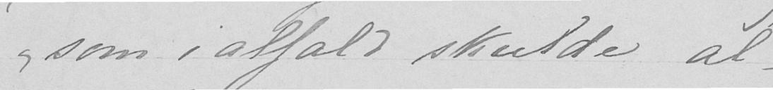som ialfald skulde al-
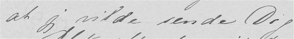at jeg vilde sende Dig
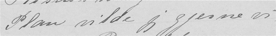Plan vilde jeg gjerne vi-
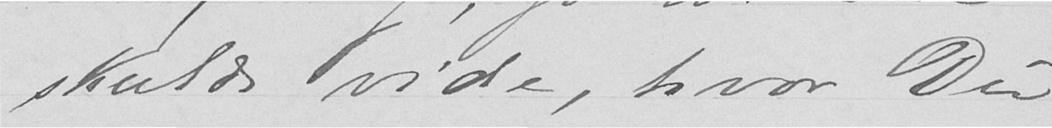skulde vide, hvor Du
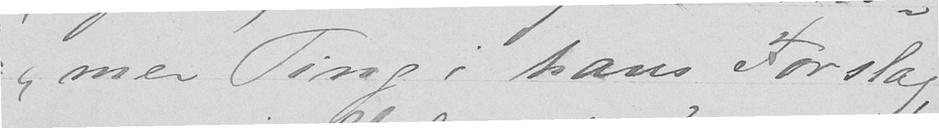mer Ting i hans Forslag,
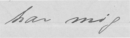har mig.
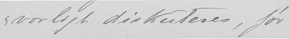vorligt diskuteres, før
